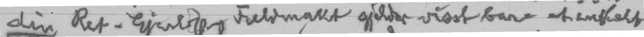din Ret. Gierløffs Fuldmakt gjelder visst bare et enkelt
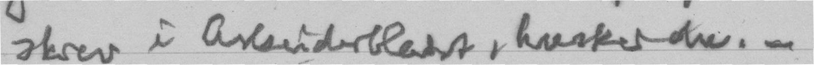skrev i Arbeiderbladet, husker du. -
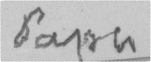Papa
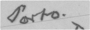Porto.
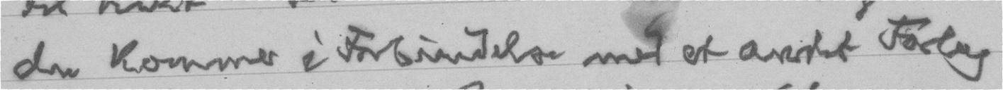du kommer i Forbindelse med et andet Forlag
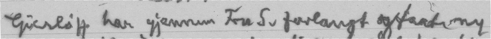Gierløff har gjennem Fru S. forlangt og faat ny
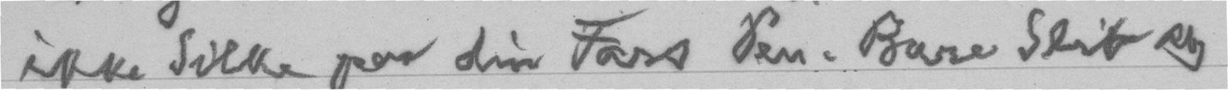ikke Silke paa din Fars Pen. Bare Slit og
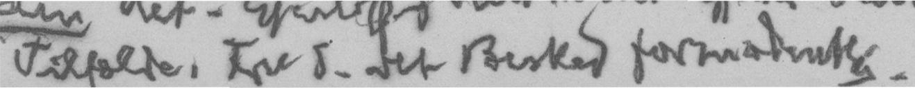Tilfælde, Fru S. vet Besked formodentlig.
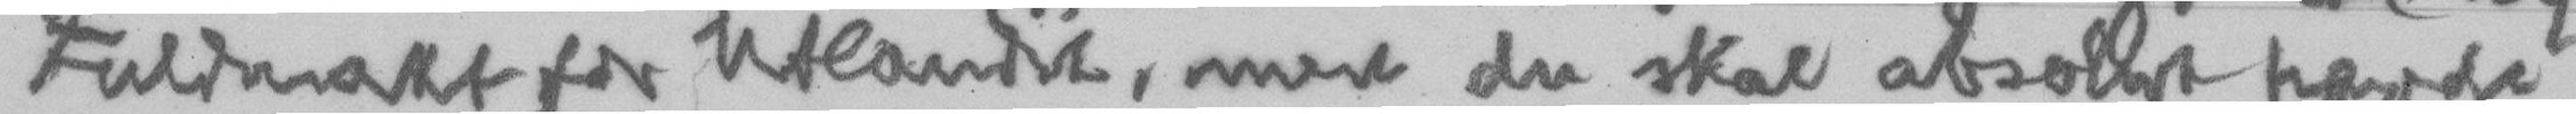Fuldmakt for Utlandet, men du skal absolut hævde
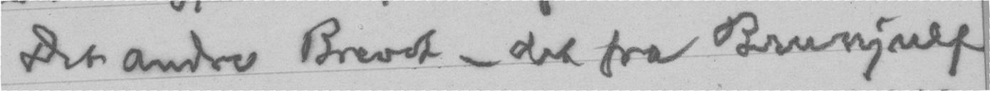Det andre Brevet - det fra Brunjulf
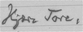Kjære Tore,
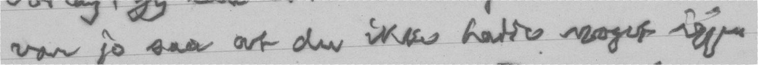var jo saa at du ikke hadde noget igjen
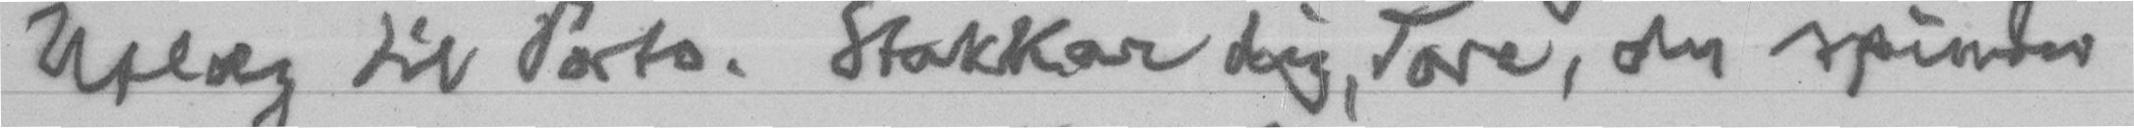Utlæg til Porto. Stakkar dig, Tore, du spinder
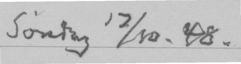Søndag 17/10. 48.
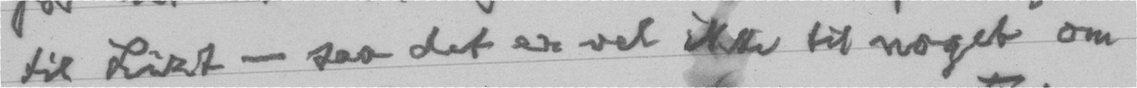til Lizt - saa det er vel ikke til noget om
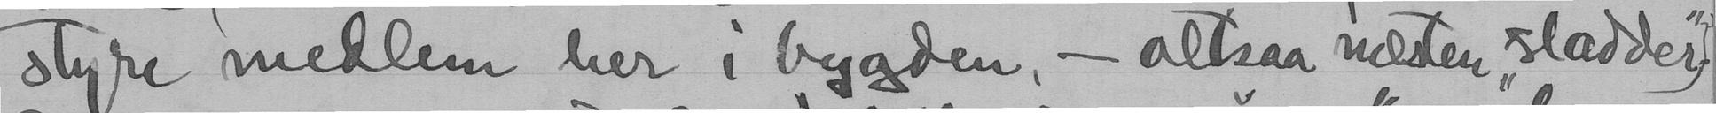styre medlem her i bygden, - altsaa næsten "sladder".
